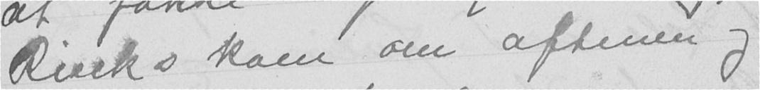Riecks kom om aftenen og
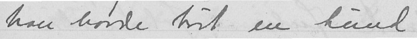han havde hørt en hund
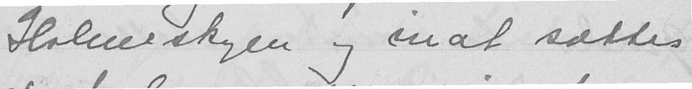Holmeskogen og inat sættes
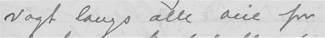vagt langs alle veie for
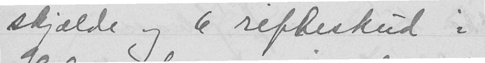skjælde og 6 rifleskud i
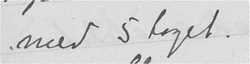med 5 toget.
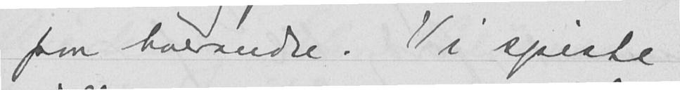paa hverandre. Vi spiste
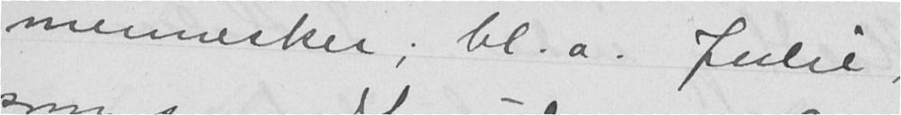mennesker; bl. a. Julie,
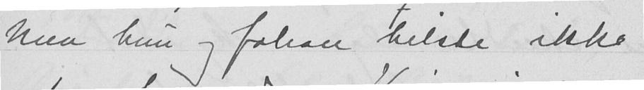Men hun og Johan hilste ikke
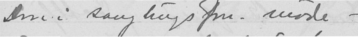Don i saugbrugsforn. møde -
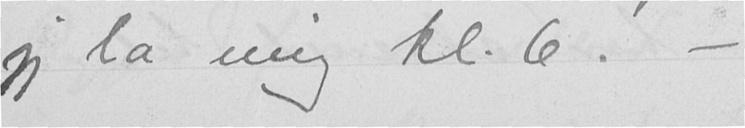jeg la mig kl. 6. -
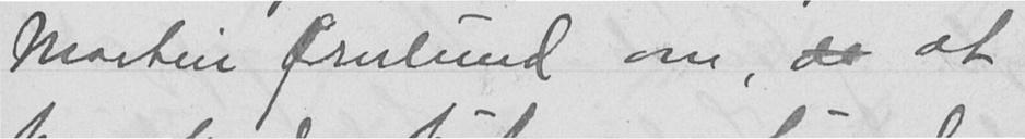Martin Ørnelund om, de at
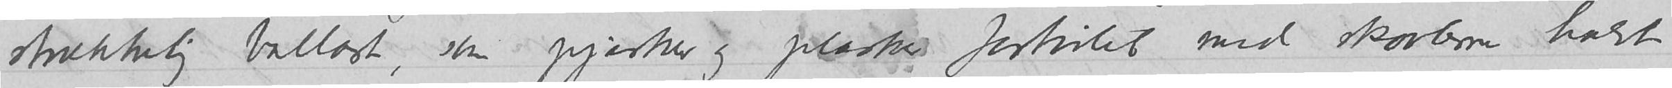strækkelig ballast, som pjasker og plasker fortvilet med skaaberne halvt
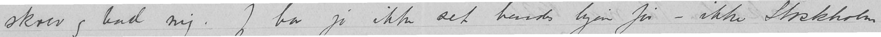skrev og bad mig.  Jeg har jo ikke set hendes hjem før – ikke Stockholm
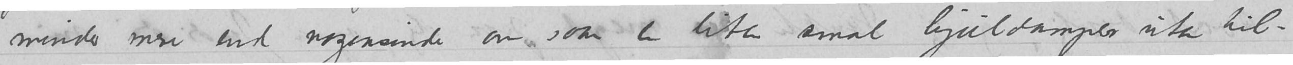minder mere end nogensinde om saan en liten smal hjuldamper uten til-
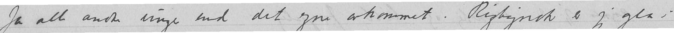for alle andre unger end det egne avkommet.  Rigtignok er jeg gla i
